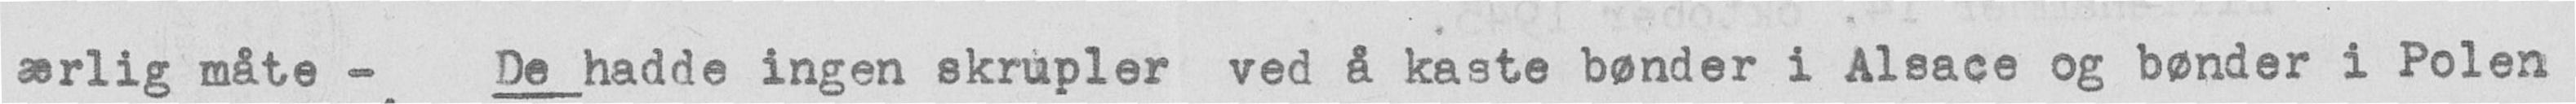ærlig måte - De hadde ingen skrupler ved å kaste bønder i Alsace og bønder i Polen
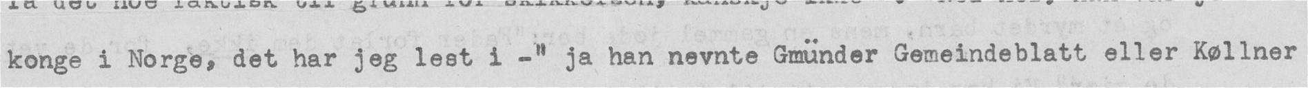konge i Norge, det har jeg lest i -" ja han nevnte Gmunder Gemeindeblatt eller Køllner
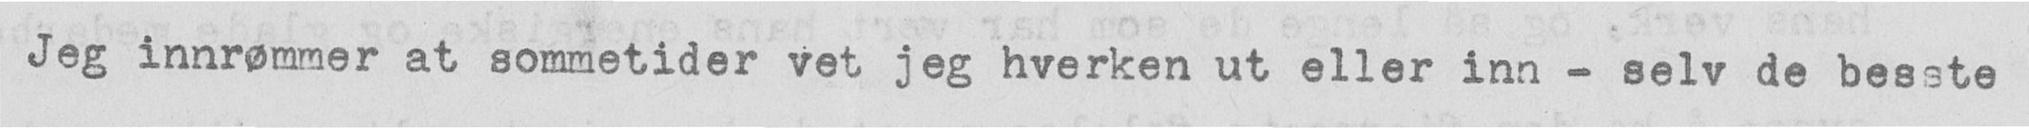Jeg innrømmer at sommetider vet jeg hverken ut eller inn - selv de besste
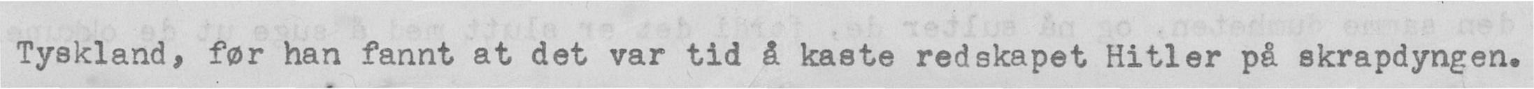Tyskland, før han fannt at det var tid å kaste redskapet Hitler på skrapdyngen.
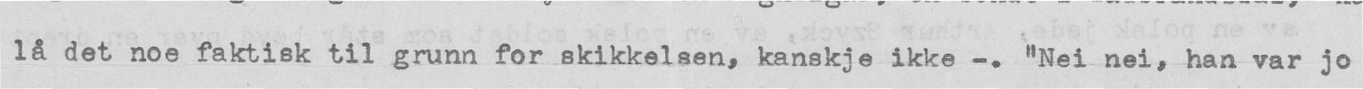lå det noe faktisk til grunn for skikkelsen, kanskje ikke -. "Nei nei, han var jo
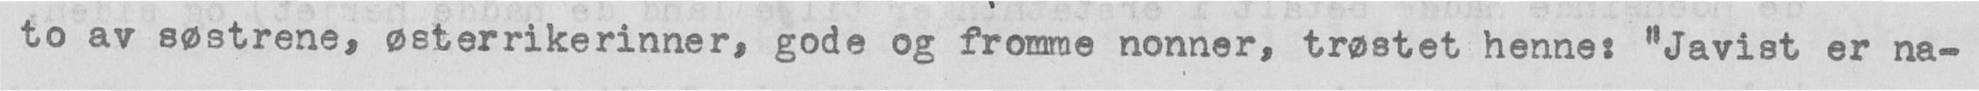to av søstrene, østerrikerinner, gode og fromme nonner, trøstet henne: "Javist er na-
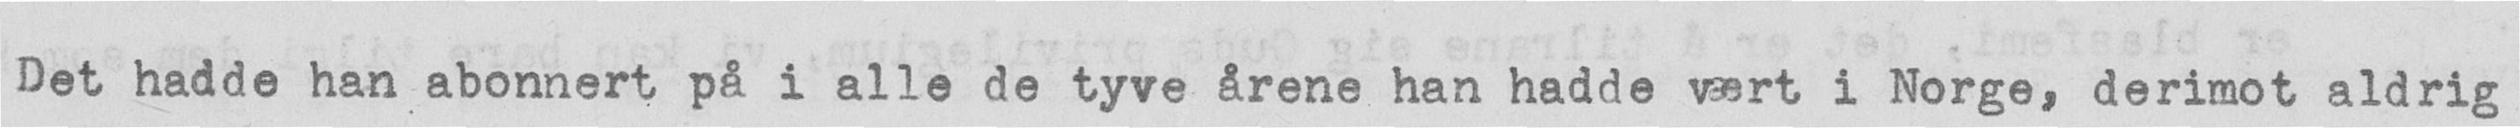Det hadde han abonnert på i alle de tyve årene han hadde vært i Norge, derimot aldrig
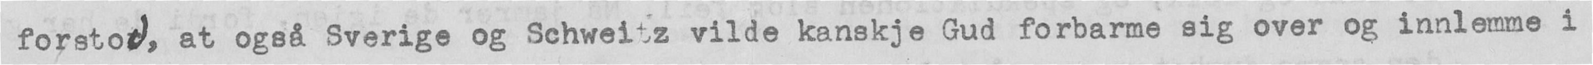forstod, at ogsa Sverige og Schweitz vilde kanskje Gud forbarme sig over og innlemme i
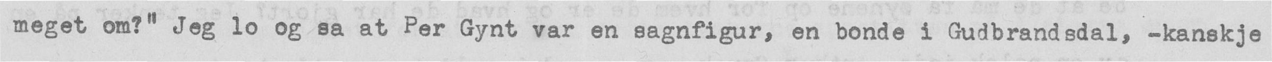meget om?" Jeg lo og sa at Per Gynt var en sagnfigur, en bonde i Gudbrandsdal, -kanskje
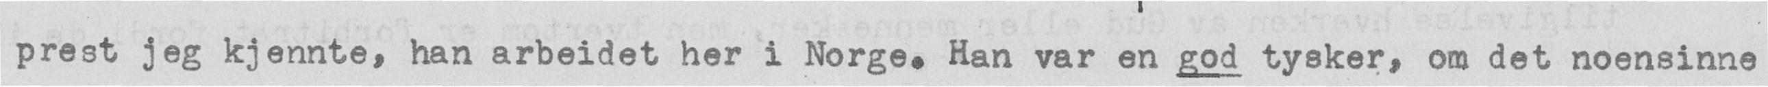prest jeg kjennte, han arbeidet her i Norge. Han var en god tysker, om det noensinne
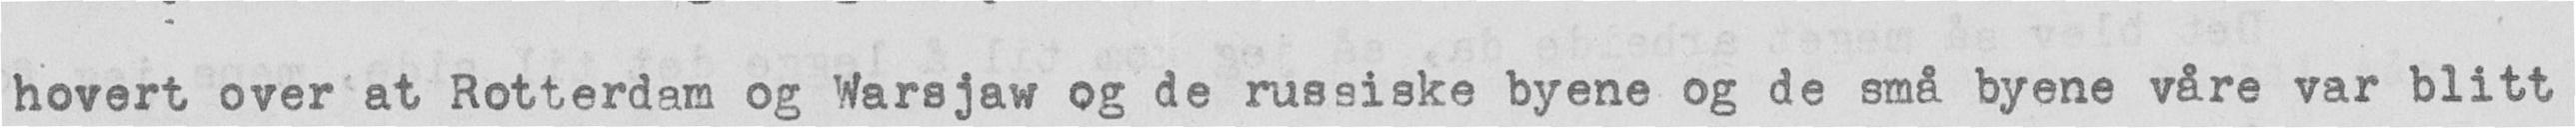hovert over at Rotterdam og Warsjaw og de russiske byene og de små byene våre var blitt
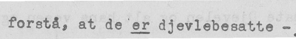forstå, at de er djevlebesatte -
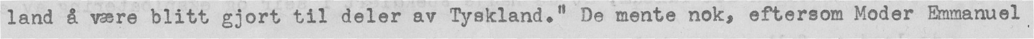land å være blitt gjort til deler av Tyskland." De mente nok, eftersom Moder Emmanuel
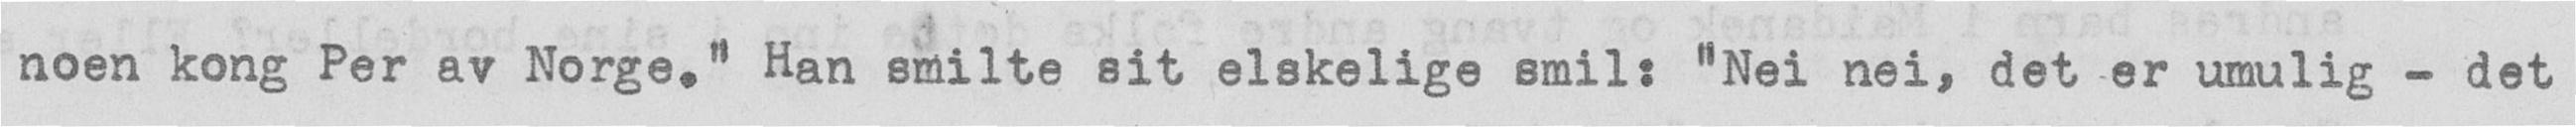noen kong Per av Norge." Han smilte sit elskelige smil: "Nei nei, det er umulig - det
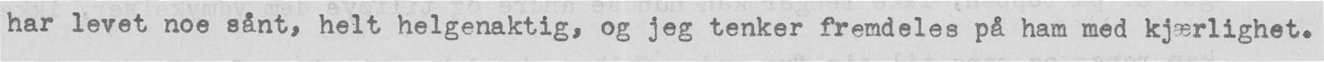har levet noe sånt, helt helgenaktig, og jeg tenker fremdeles på ham med kjærlighet.
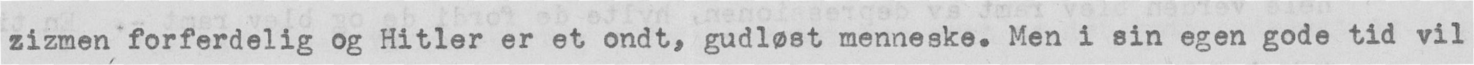zizmen forferdelig og Hitler er et ondt, gudløst menneske. Men i sin egen gode tid vil
